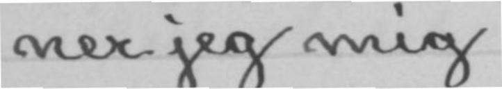ner jeg mig
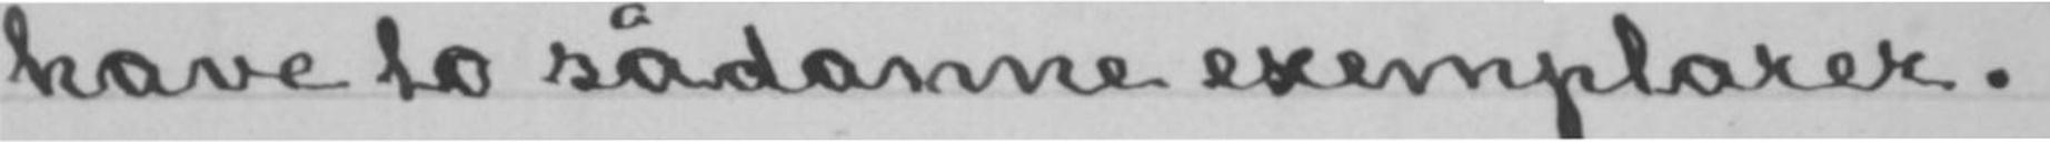have to sådanne exemplarer.
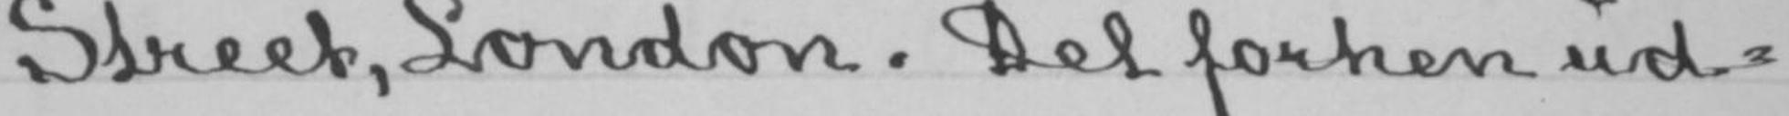Street, London. Det forhen ud-
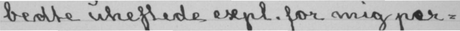bedte uheftede expl. for mig per-
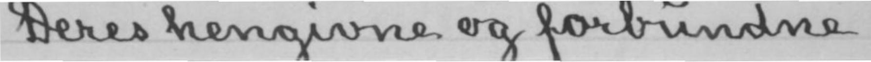Deres hengivne og forbundne
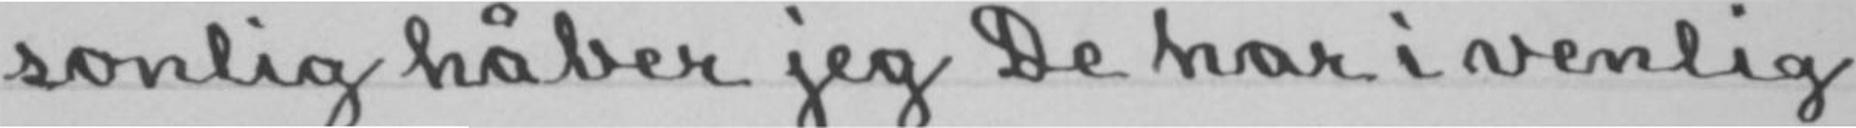sonlig håber jeg De har i venlig
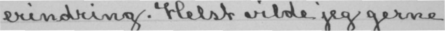erindring. Helst vilde jeg gerne
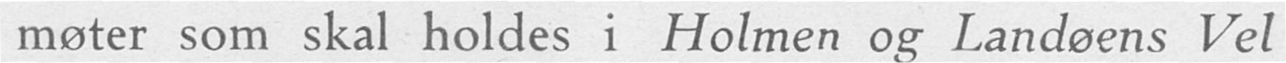møter som skal holdes i Holmen og Landøens Vel
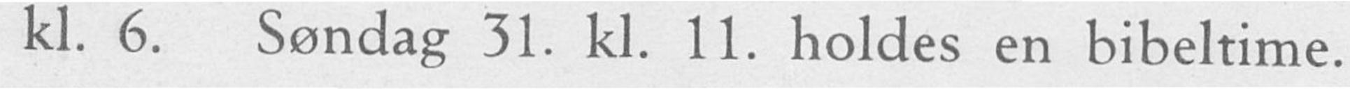kl. 6. Søndag 31. kl. 11. holdes en bibeltime.
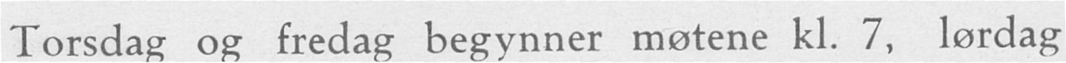Torsdag og fredag begynner møtene kl. 7, lørdag
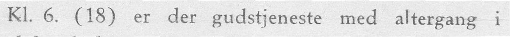Kl. 6. (18) er der gudstjeneste med altergang 1
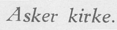Asker kirke.
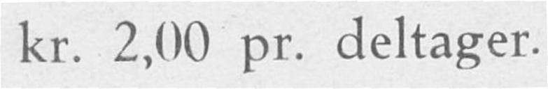kr. 2,00 pr. deltager.
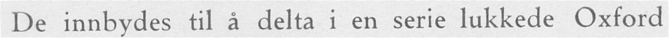De innbydes til å delta i en serie lukkede Oxford
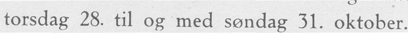torsdag 28. til og med søndag 31. oktober.
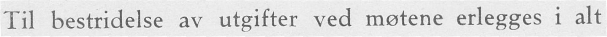Til bestridelse av utgifter ved møtene erlegges i alt
